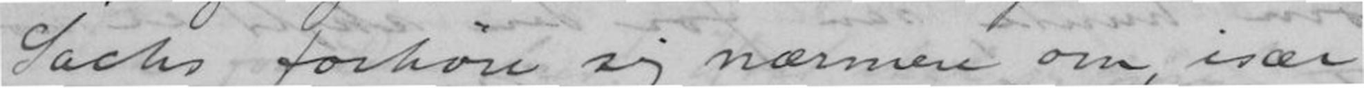Sachs forhøre sig nærmere om, især
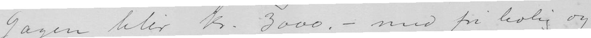Gagen blir 3000.- mnd fri bolig og
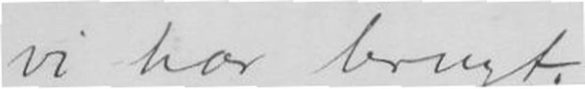vi har brugt.
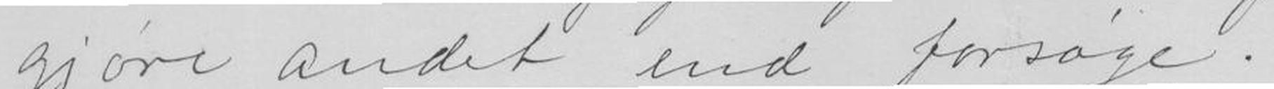gjøre andet end forsøge.
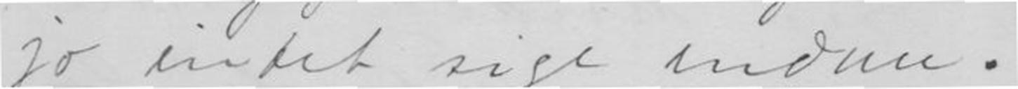jo intet sige endnu.
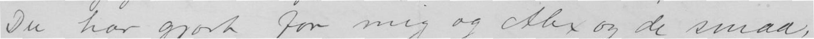Du har gjort for mig og Alex og de smaa,
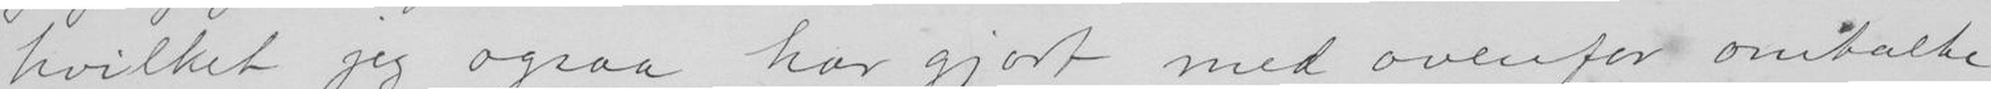hvilket jeg ogsaa har gjort med ovenfor omtalte
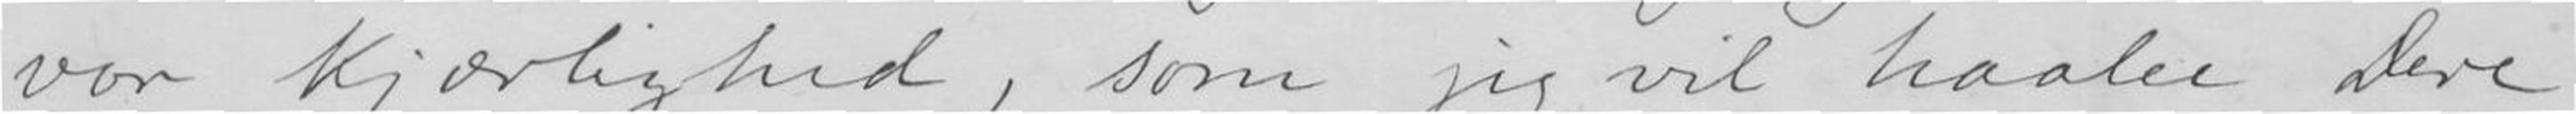vor kjærlighed, som jeg vil haabe Dere
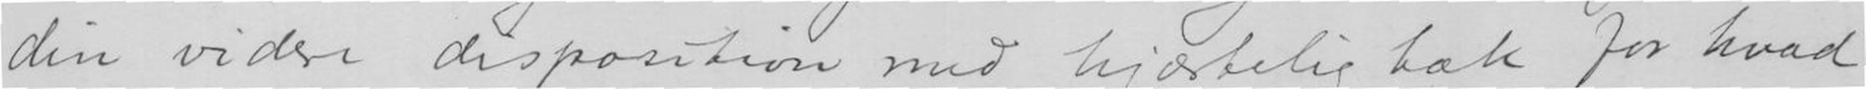din videre disposition med hjærtelig tak for hvad
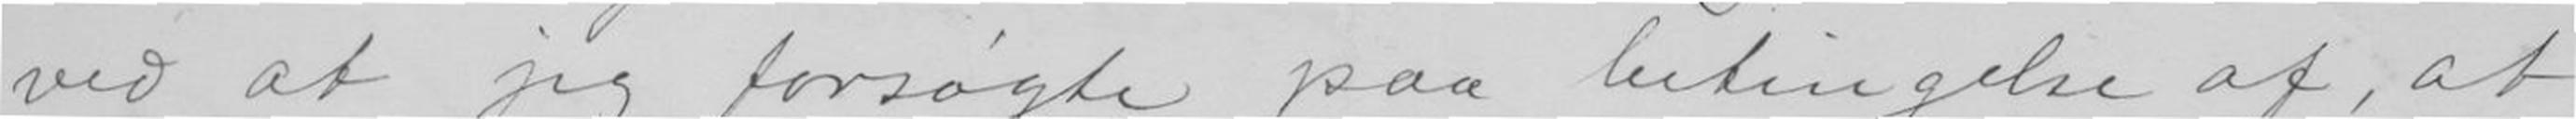ved at jeg forsøgte paa betingelse af, at
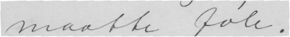maatte føle.
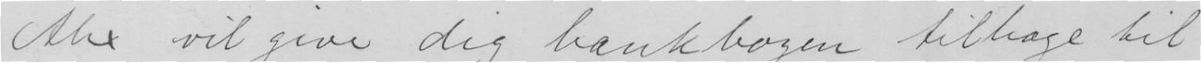Alex vil give dig bankbogen tilbage til
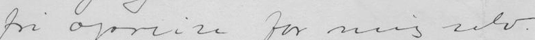fri opreise for mig selv.
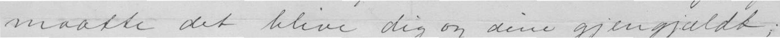maatte det blive dig og dine gjengjældt;
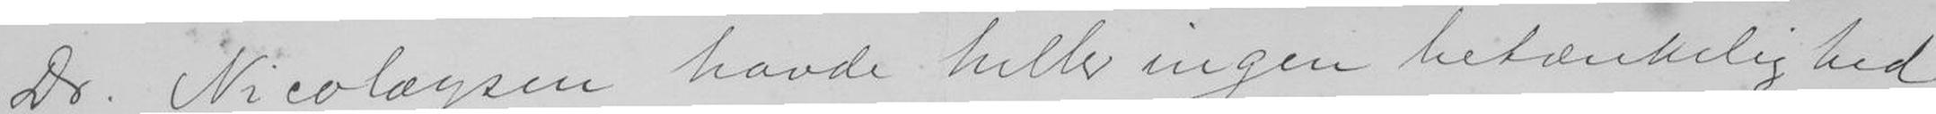Dr. Nicolaysen havde heller ingen betænkelighed
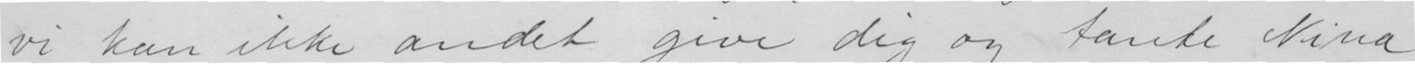vi kan ikke andet give dig og tante Nina
